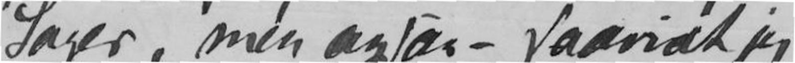Sager, men ogsaa - saavidt jeg
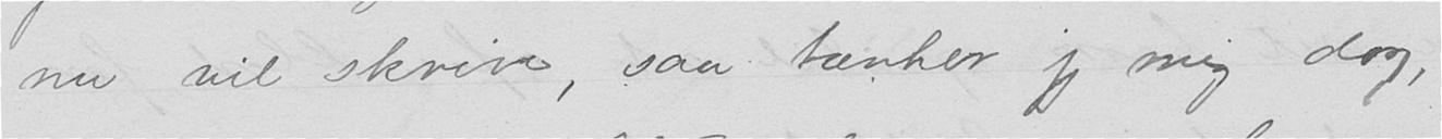nu vil skrive, saa tænker jeg mig dog,
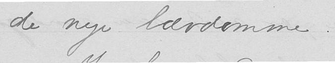de nye lærdomme.
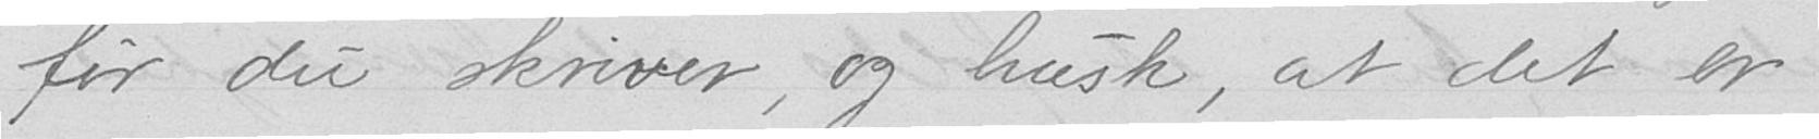før du skriver, og husk, at det er
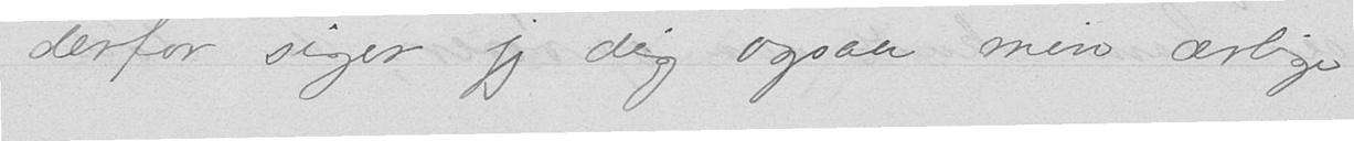derfor siger jeg dig ogsaa min ærlige
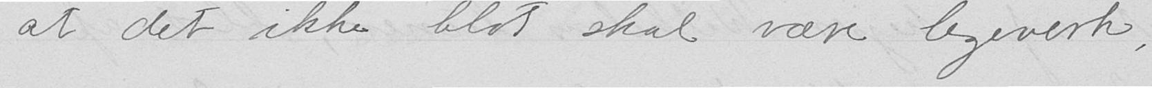at det ikke blot skal være legeverk,
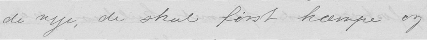de nye, de skal først kæmpe og
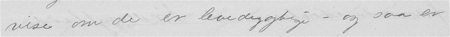vise om de er levedyktige - og saa er
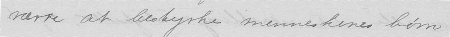værre at bestyrke menneskenes børn
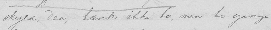skyld, Dea, tænk ikke to, men ti gange
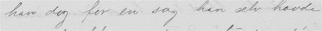han dog for en sag han selv havde
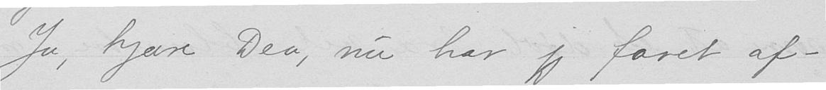Ja, kjære Dea, nu har jeg faret af-
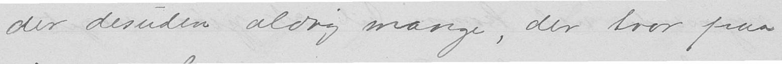der desuden aldrig mange, der tror paa
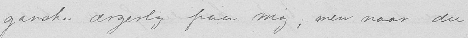ganske ærgerlig paa mig; men naar du
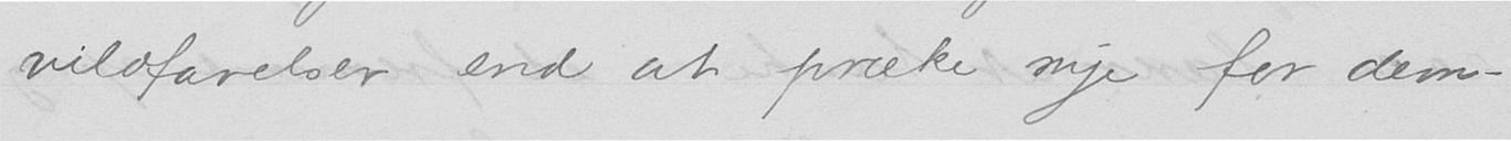vildfarelser end at præke nye for dem -
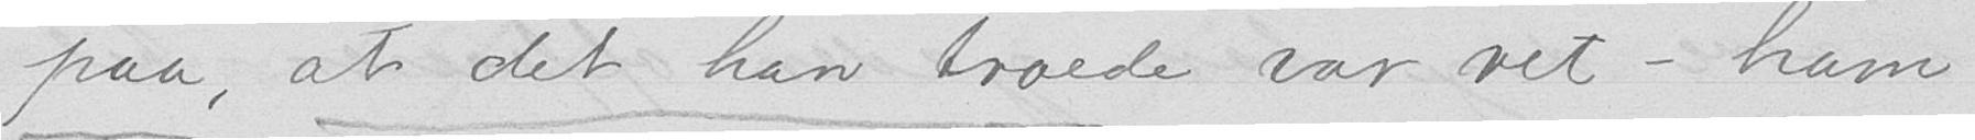paa, at det han troede var vel - ham
